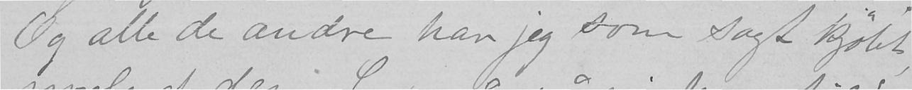Og alle de andre har jeg som sagt kjøbt,
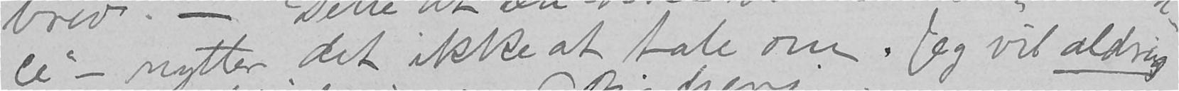ce" - nytter det ikke at tale om. Jeg vil aldrig
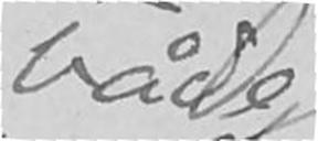både
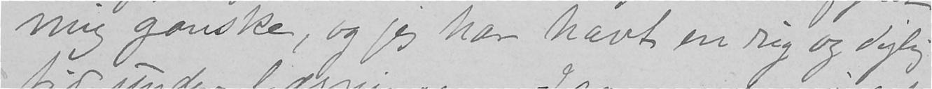mig ganske, og jeg har havt en rig og dejlig
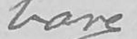bare
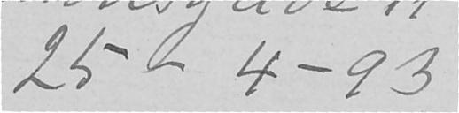25 - 4- 93
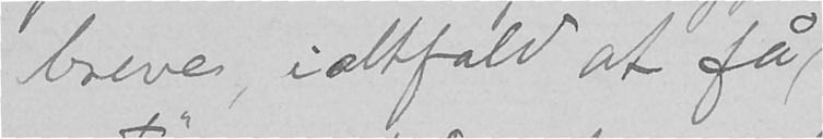breve, ialtfald at få
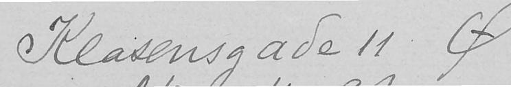Klasensgade 11 Ø
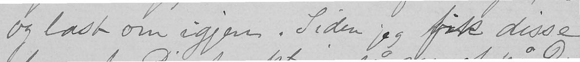og læst om igjen. Siden jeg fik disse
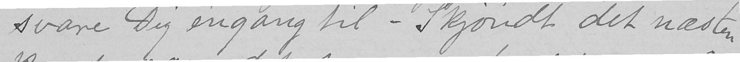svare Dig engang til - skjøndt det næsten
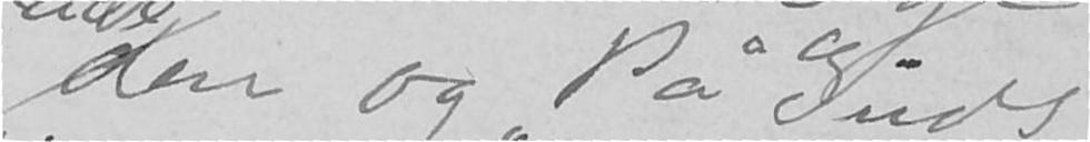den og "På Guds
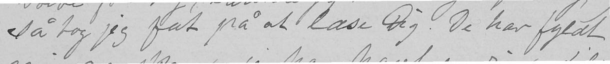så tog jeg fat på at læse Dig. De har fyldt
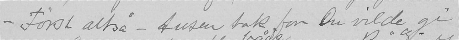- Først altså - tusen tak for Du vilde gi
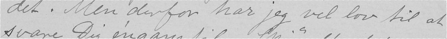det. Men derfor har jeg vel lov til at
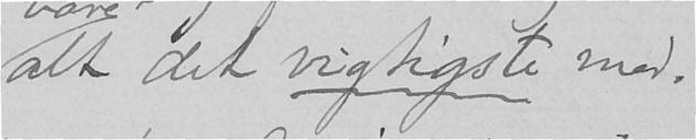alt det vigtigste med.
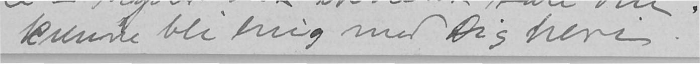kunde bli enig med Dig heri.
